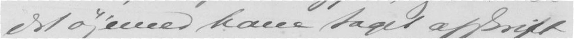det øjemed have taget afskrift
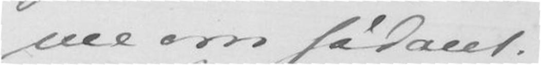me om sådant.
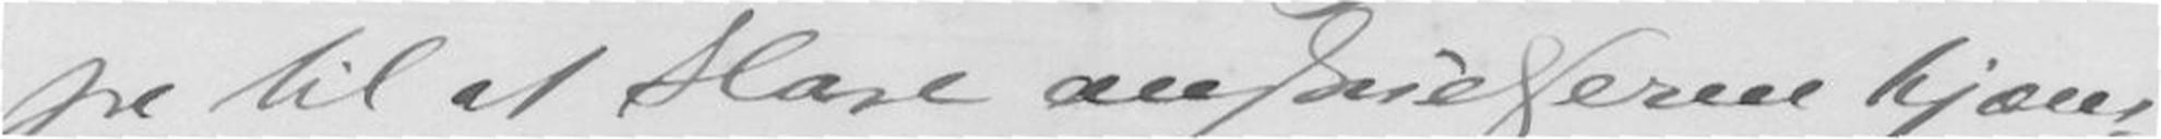pe til at klare anskuelserne hjæm-
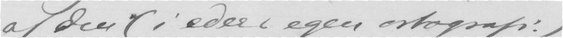af den (i eders egen ortografi!)
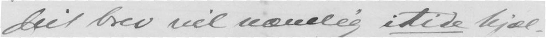Dit brev vil næmlig itide hjæl-
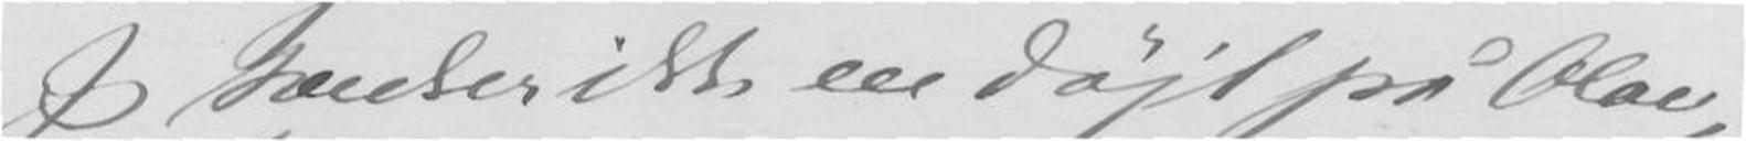Jeg tænker ikke en døjt på Olav,
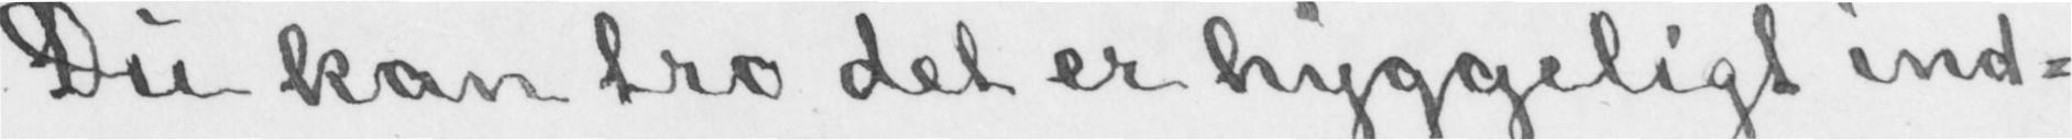Du kan tro det er hyggeligt ind-
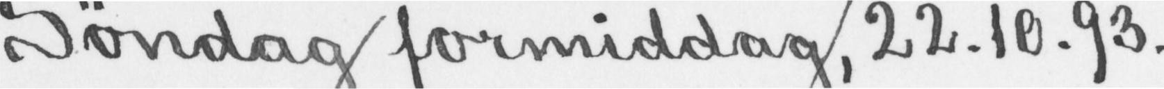Søndag formiddag, 22.10.93.
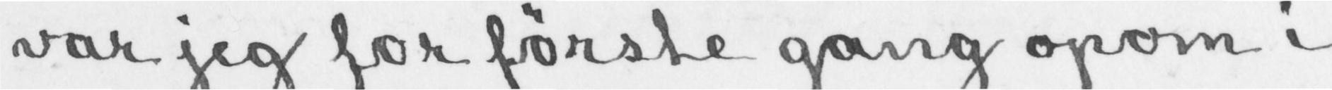var jeg for første gang opom i
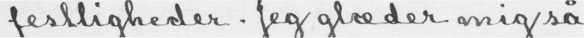festligheder. Jeg glæder mig så
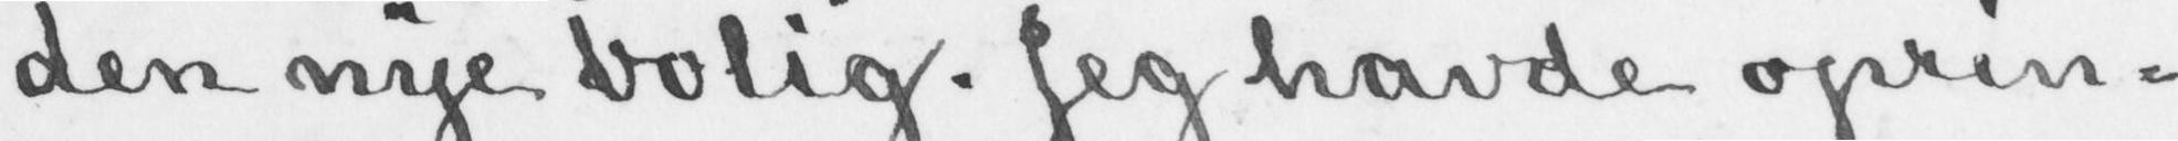den nye bolig. Jeg havde oprin-
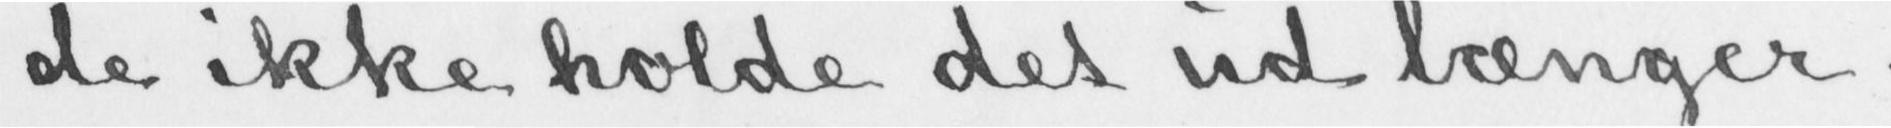de ikke holde det ud længer.
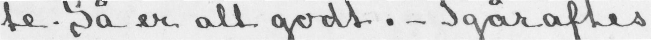te. Så er alt godt.- Igåraftes
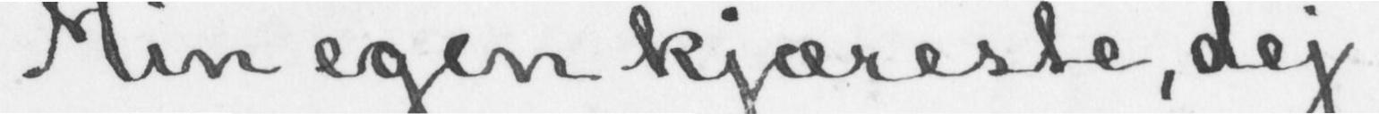Min egen kjæreste, dej-
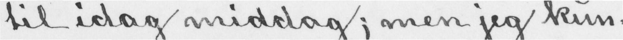til idag middag; men jeg kun-
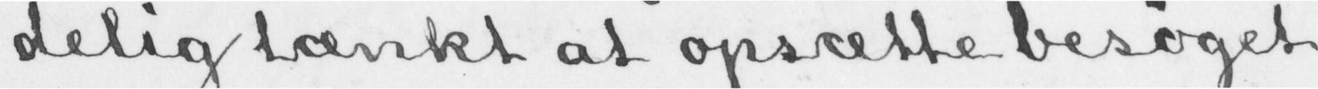delig tænkt at opsætte besøget
